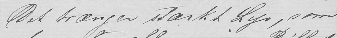Det trænger stærkt Lys, som
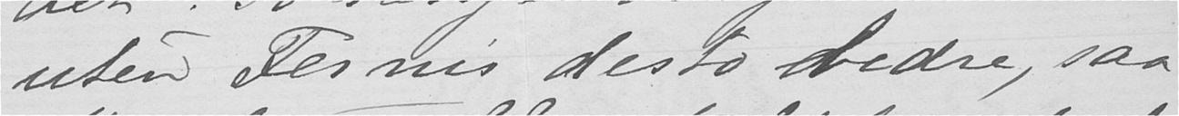uten Fernis desto bedre, saa
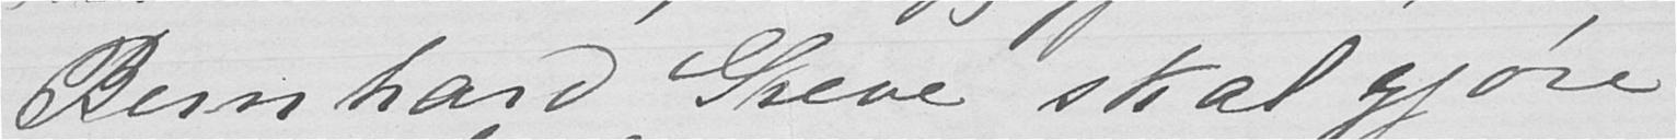Bernhard Greve skal gjøre
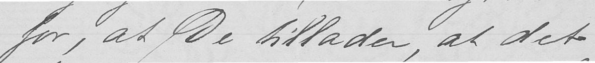for, at De tillader, at det
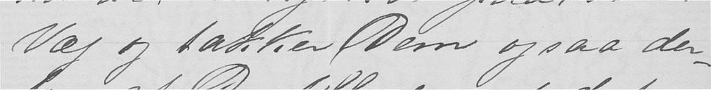Væg og takker Dem, ogsaa der-
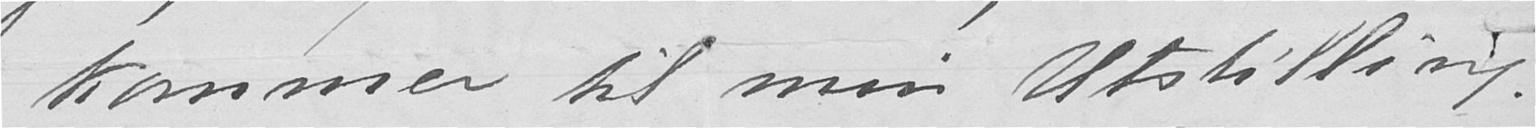kommer til min Utstilling.
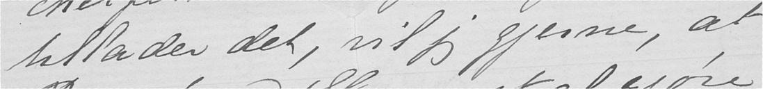tillader det, vil jeg gjerne, at
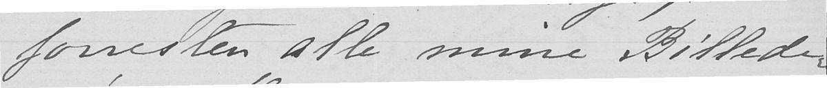forresten alle mine Billeder
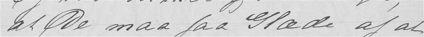at De maa faa Glæde af at
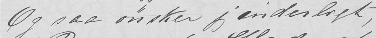Og saa ønsker jeg inderligt,
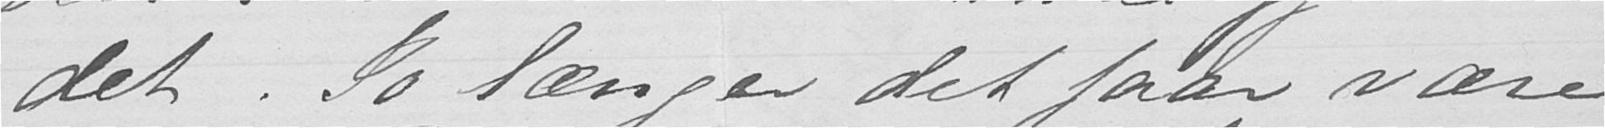det. Jo længer det faar være
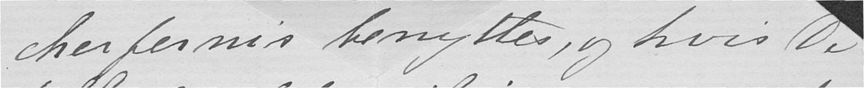cherfernis benyttes, og hvis De
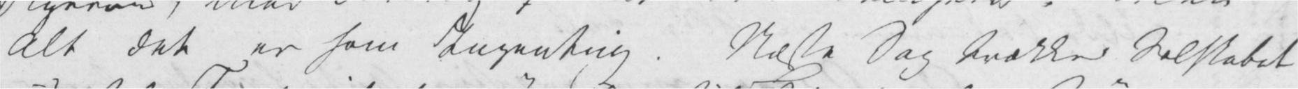Alt det er som Ingenting. Næste Dag trækker Selskabet
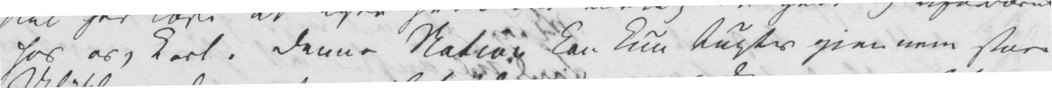hos os, Carl. Denne Nation kan kun tugtes gjennem store
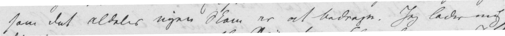som det aldeles ingen Skam er at bedrage. Jeg lader mig
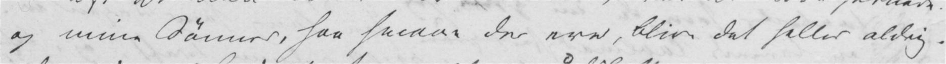og mine Sønner, saa smaae de ere, blive det heller aldrig.
